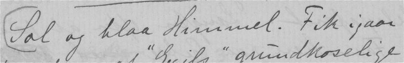Sol og blaa Himmel. Fik igaar
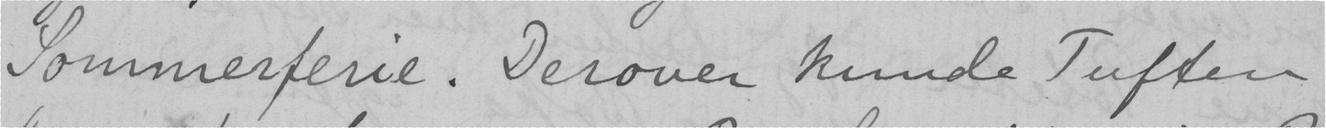Sommerferie. Derover kunde Tuften
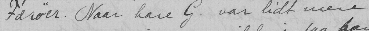Færøer. Naar bare G. var lidt mere
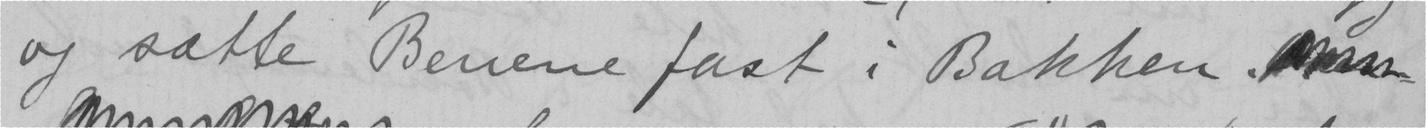og sætte Benene fast i Bakken.
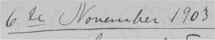6te November 1903
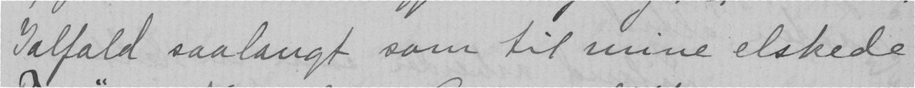Ialfald saalangt som til mine elskede
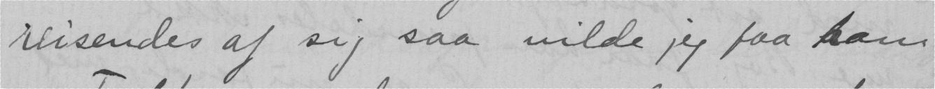reisendes af sig, saa vilde jeg faa ham
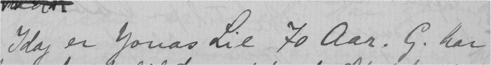Idag er Jonas Lie 70 aar. G. har
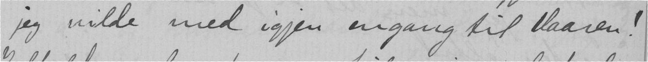jeg vilde med igjen engang til Vaaren!
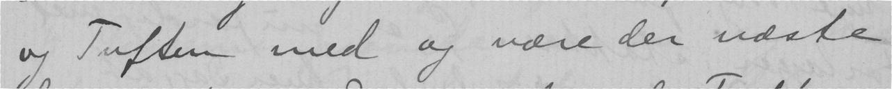og Tuften med og være der næste
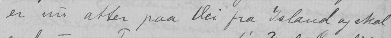er nu atter paa vei fra Island og skal
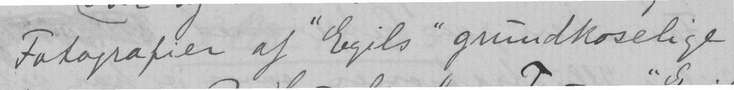Fotografier af "Egils" grundkoselige
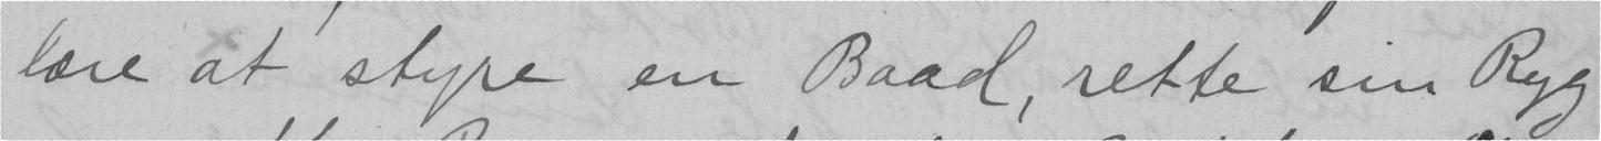lære at styre en Baad, rette sin Ryg
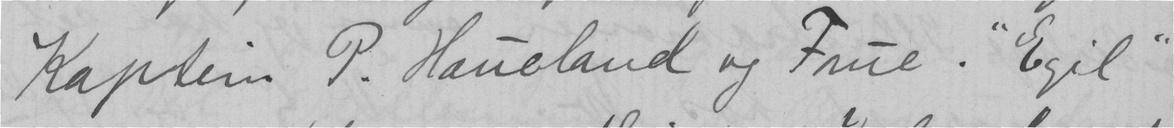Kaptein P. Haueland og Frue. "Egil"
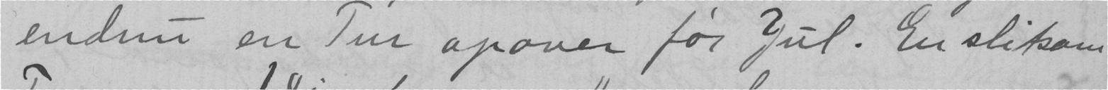endnu en Tur opover før Jul. En slitsom
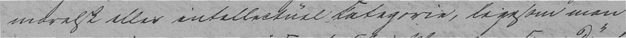moralsk eller intellectuel Kategorie, ligesom man
